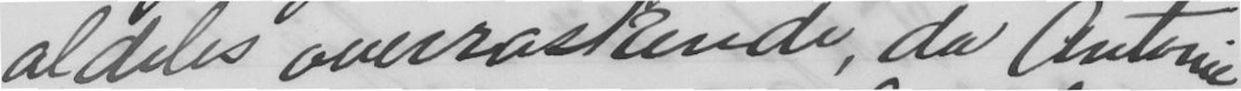aldeles overraskende, da Antonie
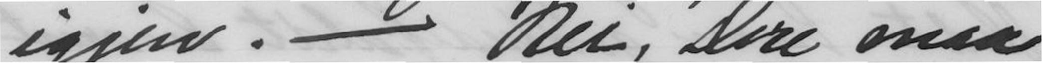igjen. - Nei, Dere maa
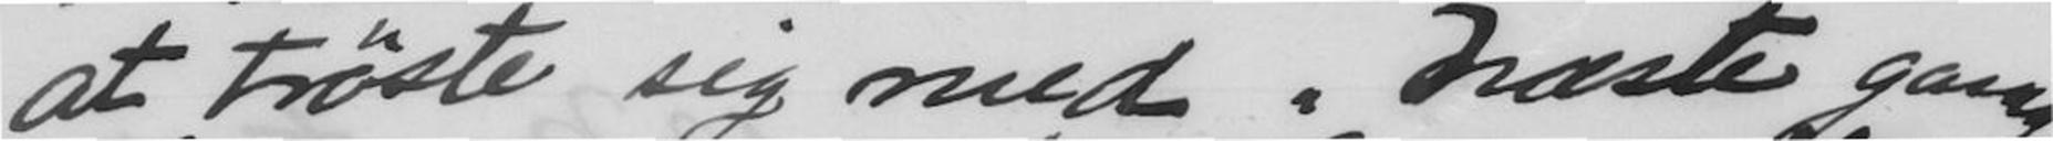at trøste sig med "Næste gang
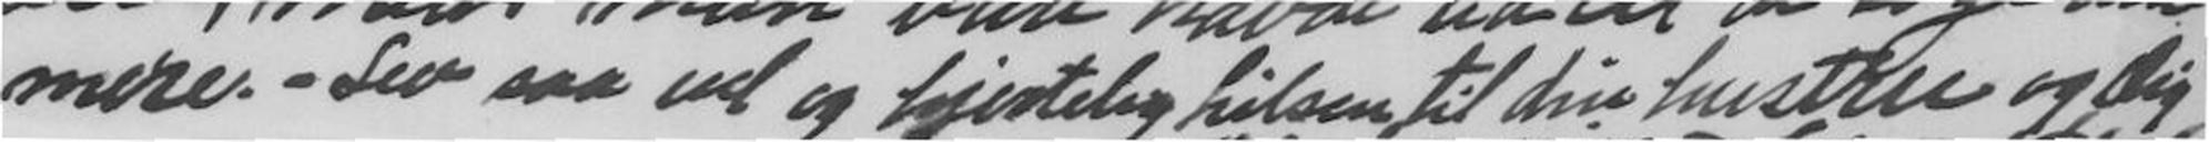mere. - Lev saa vel og hjertelig hilsen til din hustru og Dig
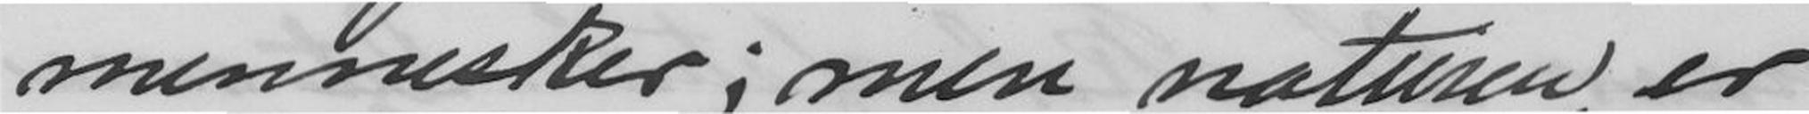mennesker; men naturen er
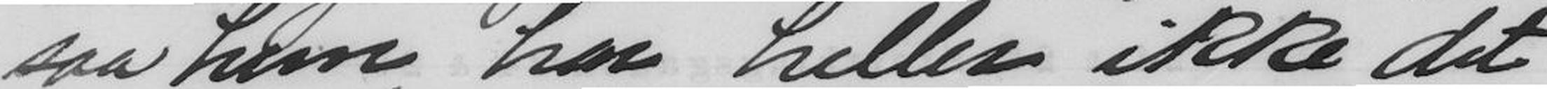saa hun har heller ikke det
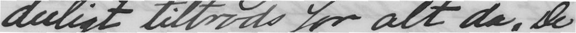deiligt tiltrods for alt da. De
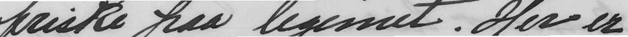friske paa legemet. Her er
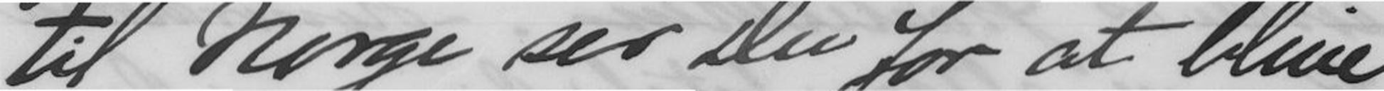til Norge ser Du for at blive
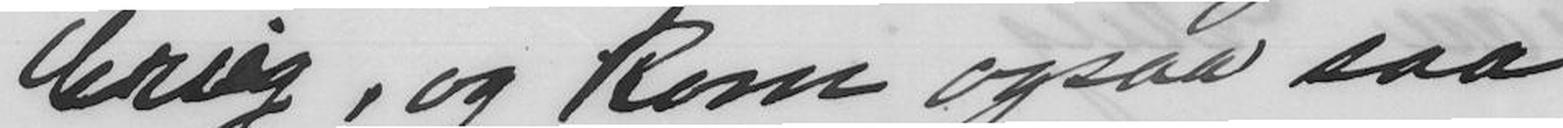Grieg, og kom ogsaa saa
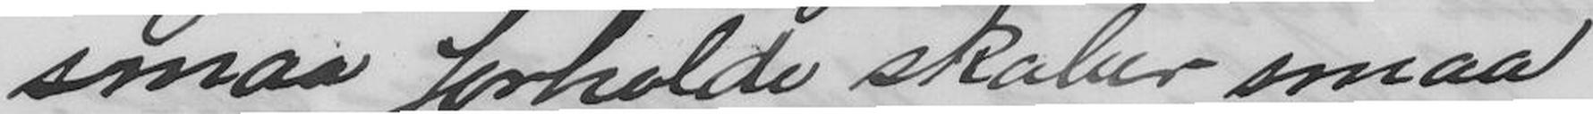smaa forholde skaber smaa
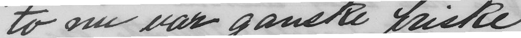to nu var ganske friske
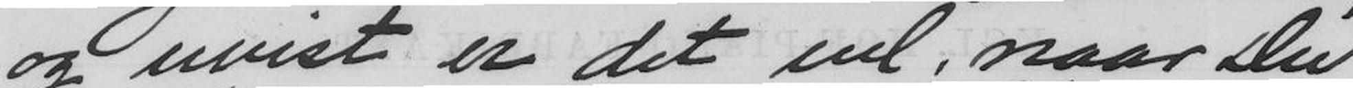og uvist er det vel, naar Du
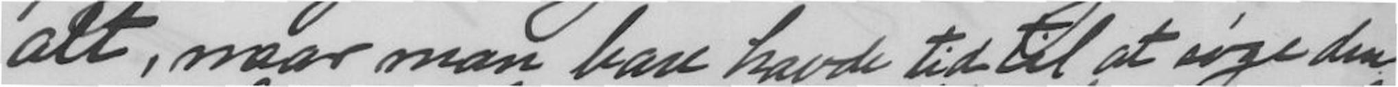alt, naar man bare havde tid til at søge den
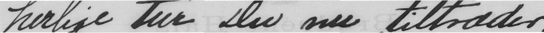herlige tur Du nu tiltræder, -
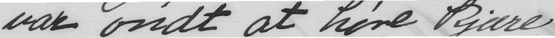var ondt at høre kjære
